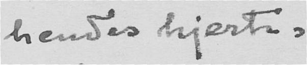hendes hjerte,
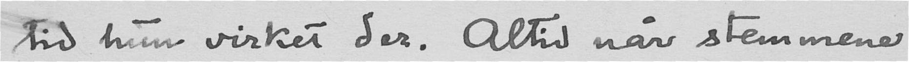tid hun virket der. Altid når stemmene
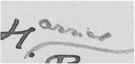Harriet
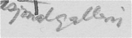asjonalgalleri
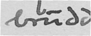brudd
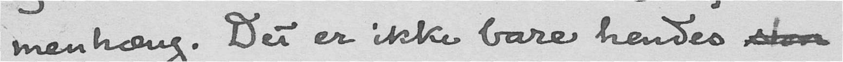menhæng. Det er ikke bare hendes store
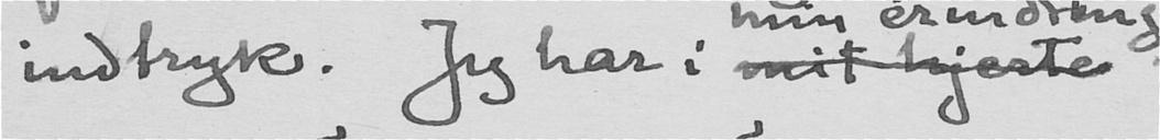indtryk. Jeg har i mit hjerte
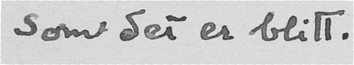som det er blitt.
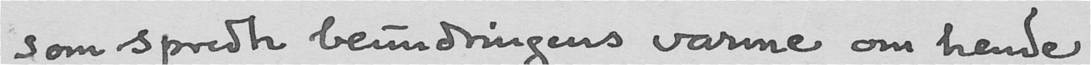som spredte beundringens varme om hende
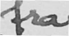fra
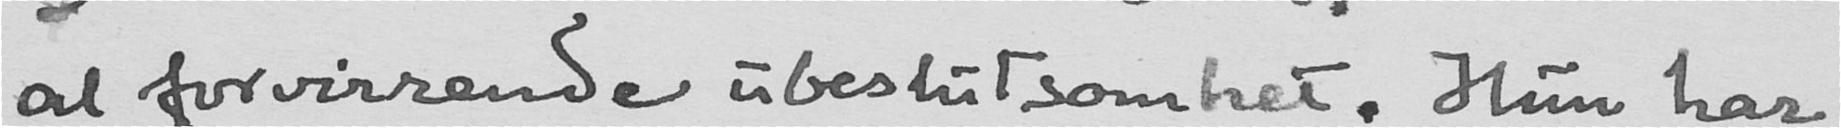al forvirrende ubeslutsomhet. Hun har
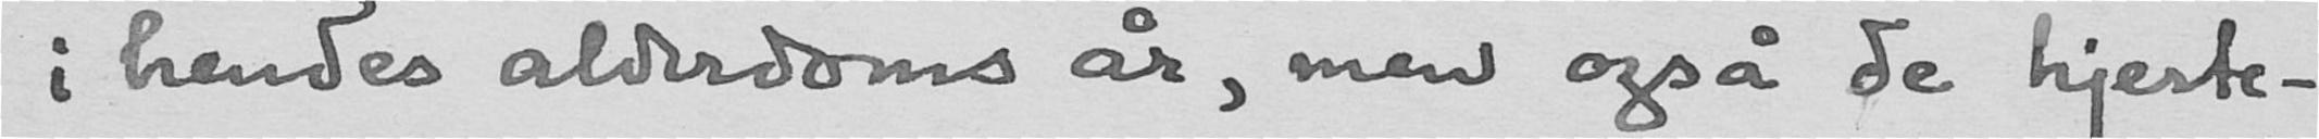i hendes alderdoms år, men også de hjerte-
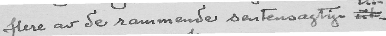flere av de rammende sentensagtige ut-
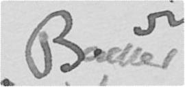Backer
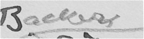Backer
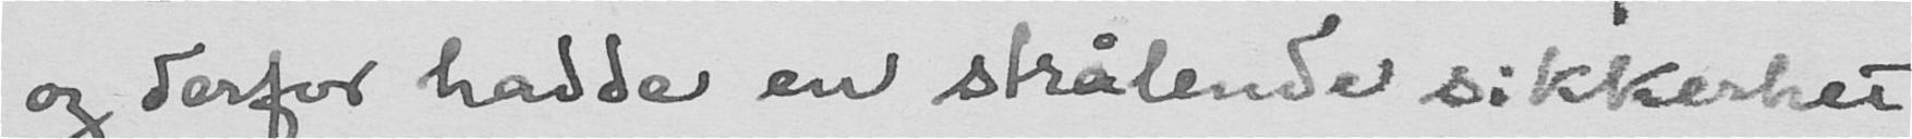og derfor hadde en strålende sikkerket
